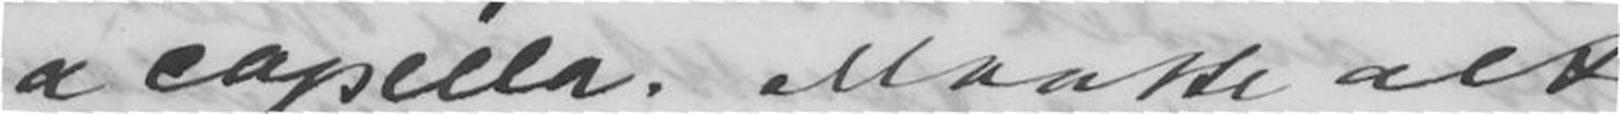a capella. Maatte alt
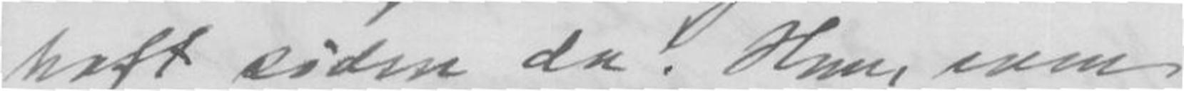haft siden da. Hun, som
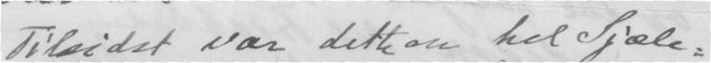Tilsidet var dette en hel Sjæle-
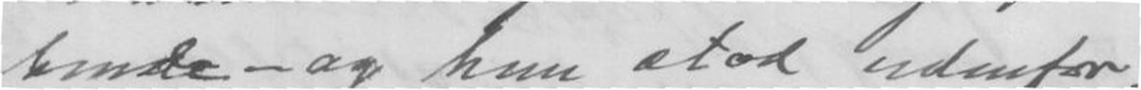hende- og hun stod udenfor!
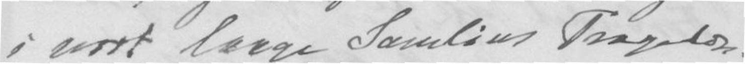i vort lange Samlivs Tragedier.
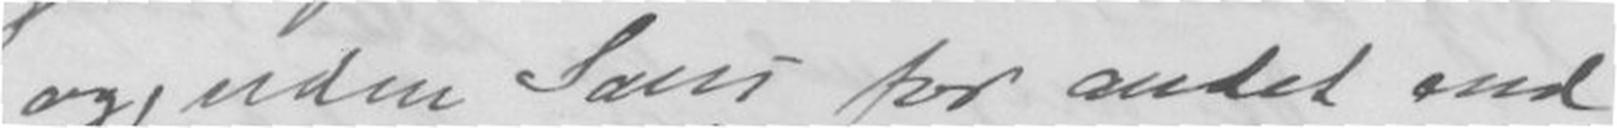og, uden Sans for andet end
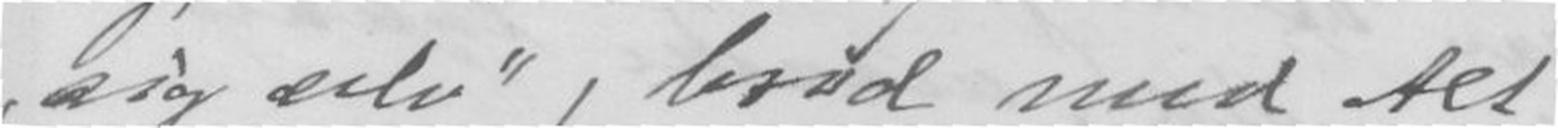"sig selv", brød med Alt
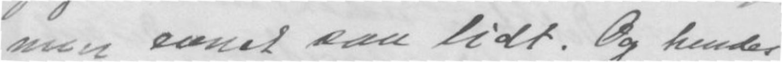men evnet saa lidt. Og hendes
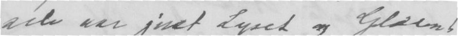selv var just Lyset og Glæden!
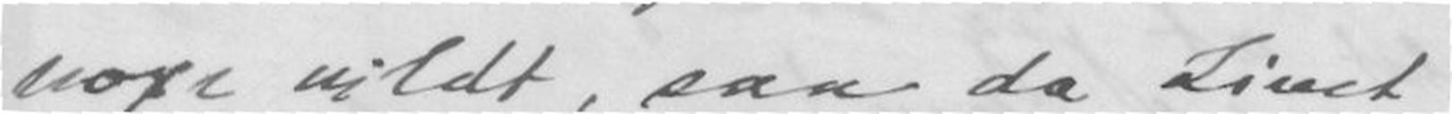voxe vildt, saa da Livet
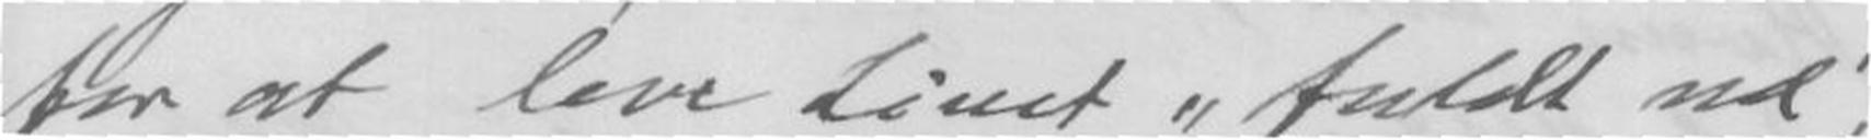for at leve Livet "fuldt ud",
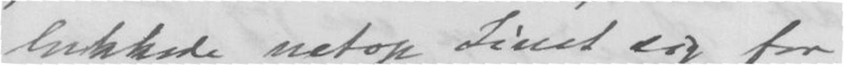lukkede netop Livet sig for
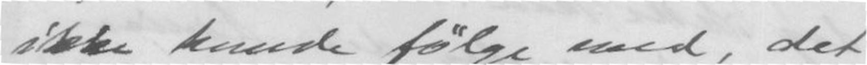ikke kunde følge med, det
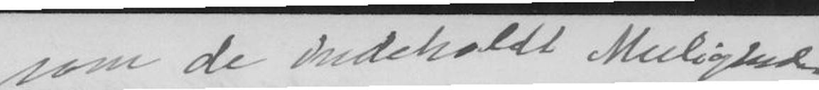som de indeholdt Muligheden
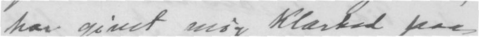har givet mig Klarhed paa
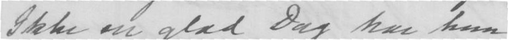Ikke en glad dag har hun
